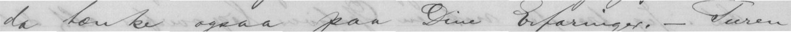da tænke ogsaa paa Dine Erfaringer. - Turen
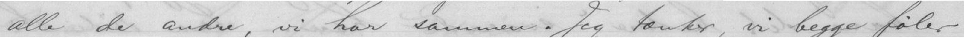alle de andre, vi har sammen. Jeg tænker, vi begge føler
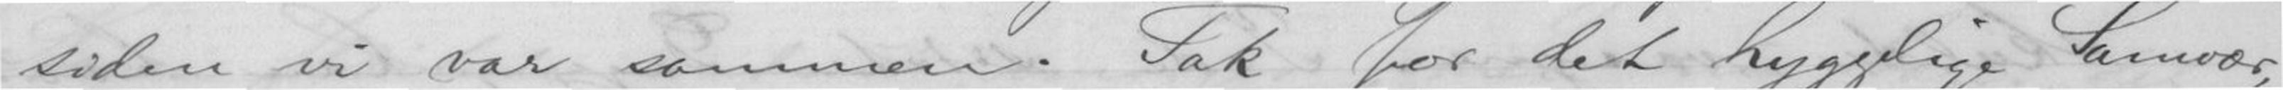siden vi var sammen. Tak for det hyggelige Samvær,
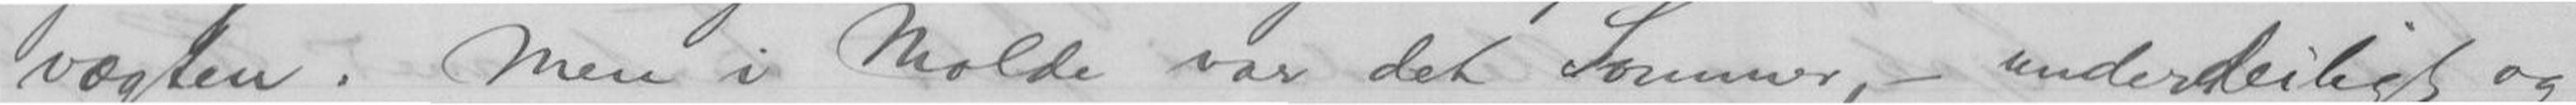vægten. Men i Molde var det Sommer, - underdeiligt og
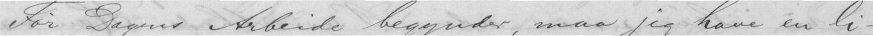Før Dagens Arbeide begynder, maa jeg have en li-
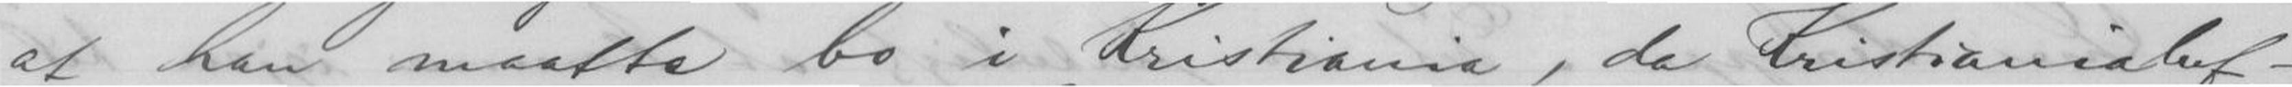at han maatte bo i Kristiania, da Kristianialuf-
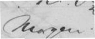Morgen.
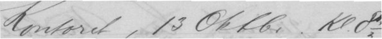Kontoret, 13 Oktbr. kl. 8½
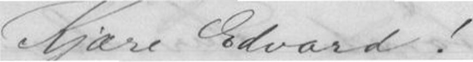Kjære Edvard!
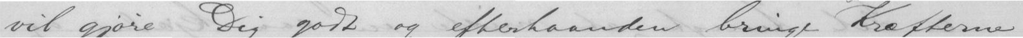vil gjøre Dig godt og efterhaanden bringe Kræfterne
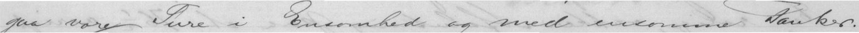gaa vore Ture i Ensomhed og med ensomme Tanker.
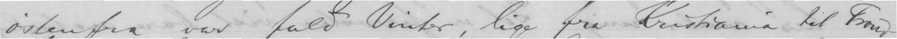østenfra var fuld Vinter, lige fra Kristiania til Trond-
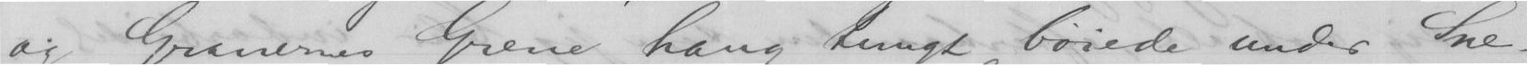og Granernes Grene hang tungt bøiede under Sne-
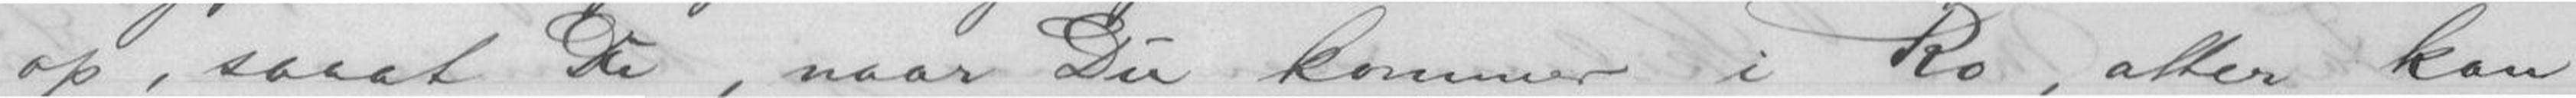op, saaat Du, naar Du kommer i Ro, atter kan
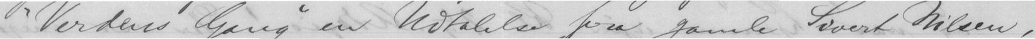"Verdens Gang" en Udtalelse fra gamle Sivert Nilsen,
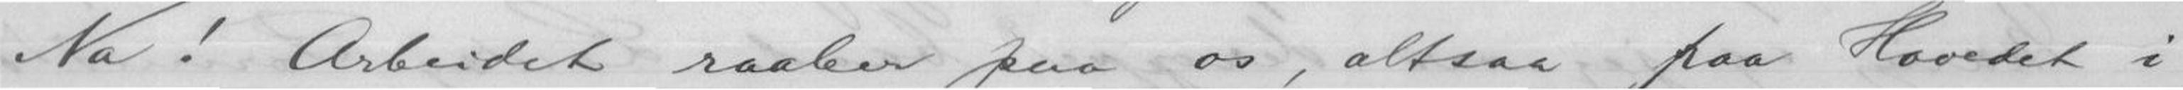Na! Arbeidet raaber paa os, altsaa paa Hovedet i
